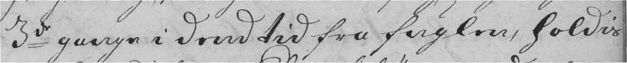3de gange i dend tid fra fuglen, holdis
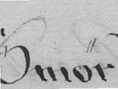Smør
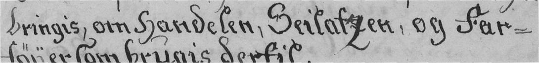bringis, om Handelen, Seilatzen, og Far-
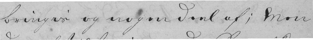bringes og nogen deel af; men
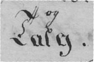Talg.
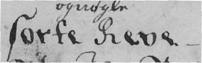sorte Reve
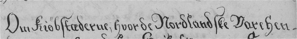Om Kiøbstæderne, hvor de Nordlandske Vare hen-
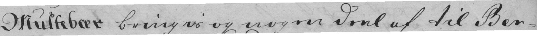Multebær bringis og nogen deel af til Ber-
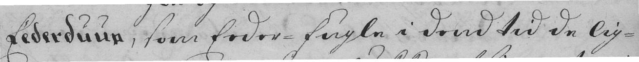Ederduun, som Feder-fugle i dend tid de lig-
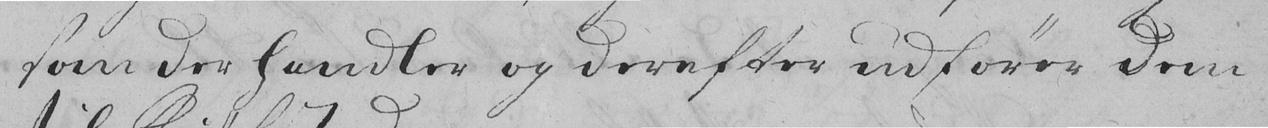som der handler og derefter udfører dem
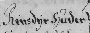Rinsdyr-huder
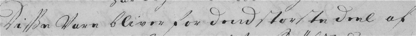Disse Vare bliver for dend største deel af
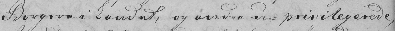Borgere i Landet, og andre u-priviligerede
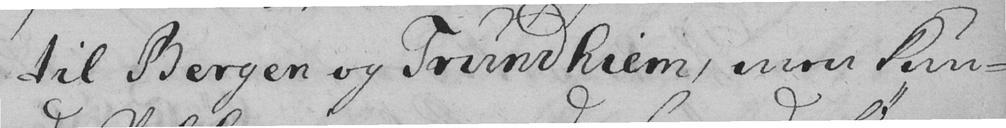til Bergen og Trundhiem, men kun-
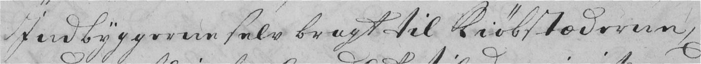Indbyggerne selv bragt til Kiøbstæderne,
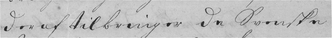der af tilbringer de Svenske
